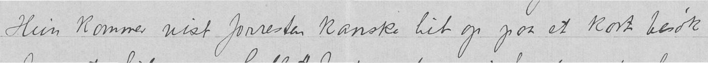Hun kommer vist forresten kanske hit op paa et kort besøk
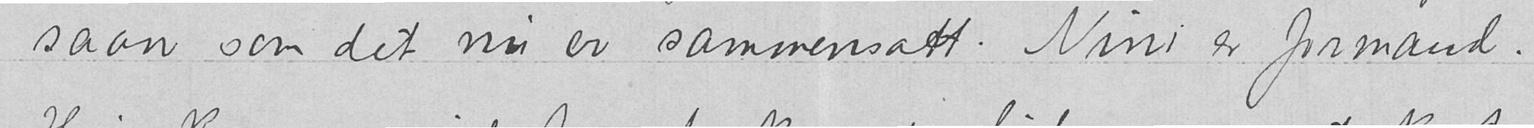saan som det nu er sammensatt. Nini er formand.
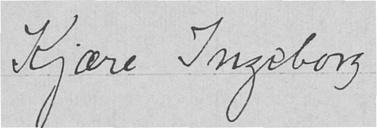Kjære Ingeborg,
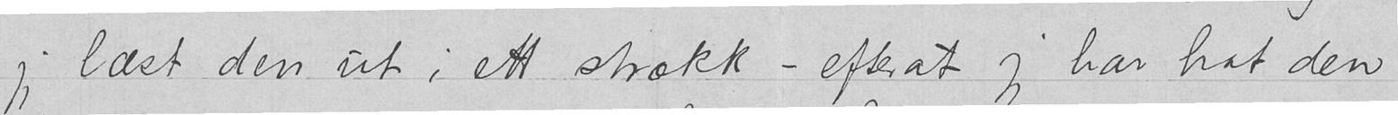jeg læst den ut i ett strækk – efterat jeg har hat den
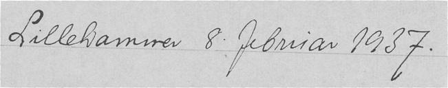Lillehammer 8. februar 1937.
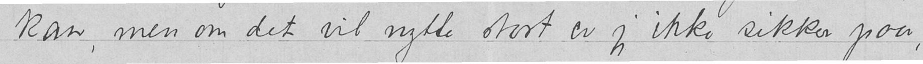kan, men om det vil nytte stort er jeg ikke sikker paa,
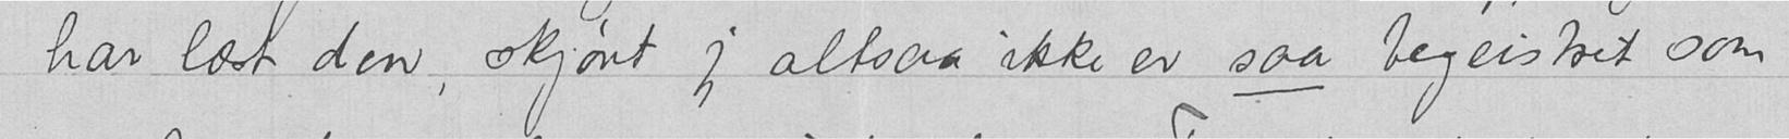jeg har læst den, skjønt jeg altsaa ikke er saa begeistret som
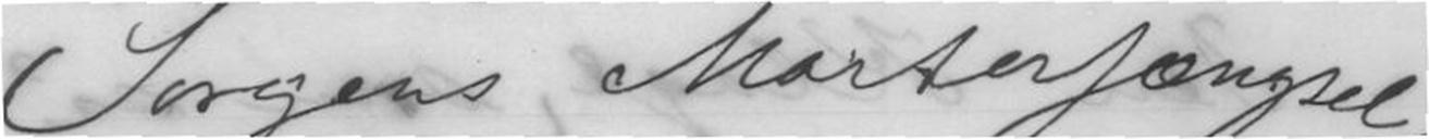Sorgens Marterfængsel!
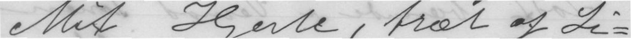Mit Hjerte, træt af Li-
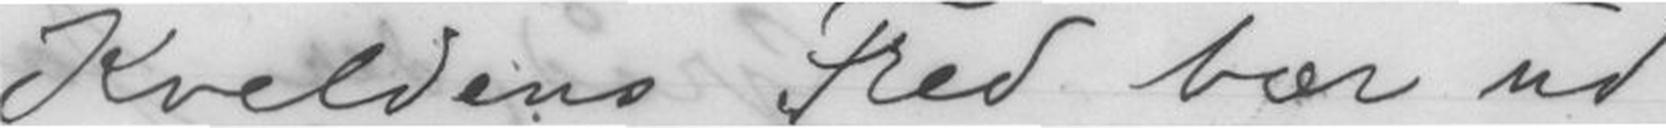Kveldens Fred bær ud
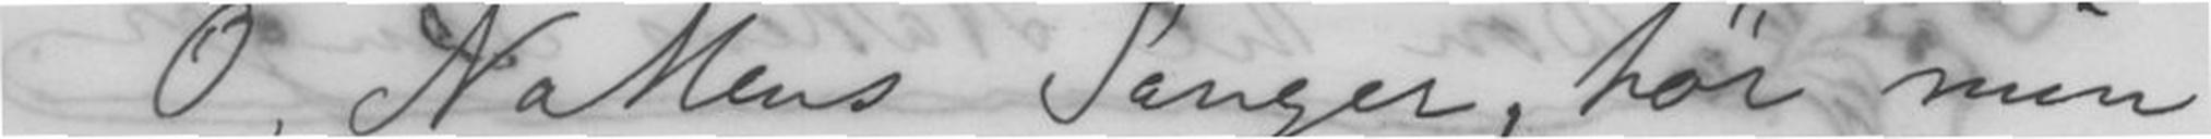O, Nattens Sanger, hør min
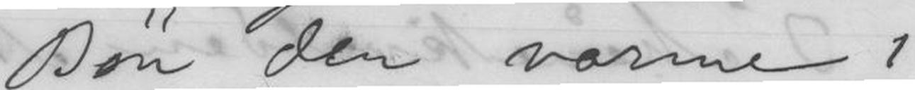Bøn den varme!
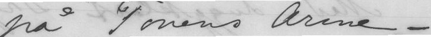på Tonens Arme. -
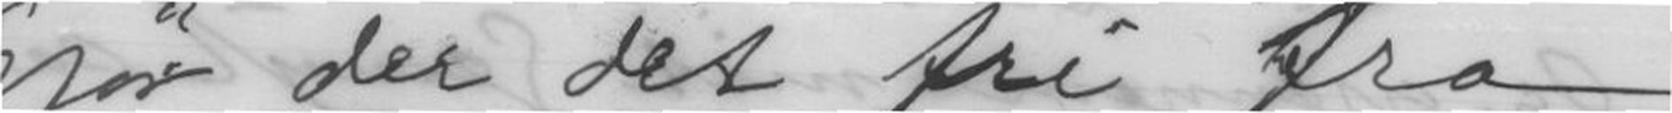Gjør der det fri fra
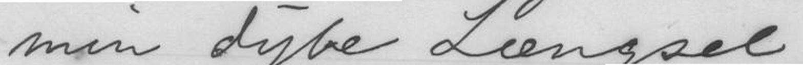min dybe Længsel!
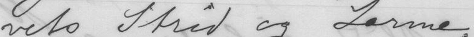vets Strid og Larme,
